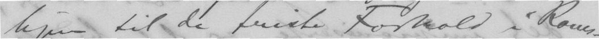hjem til de friske Forhold i Roms-
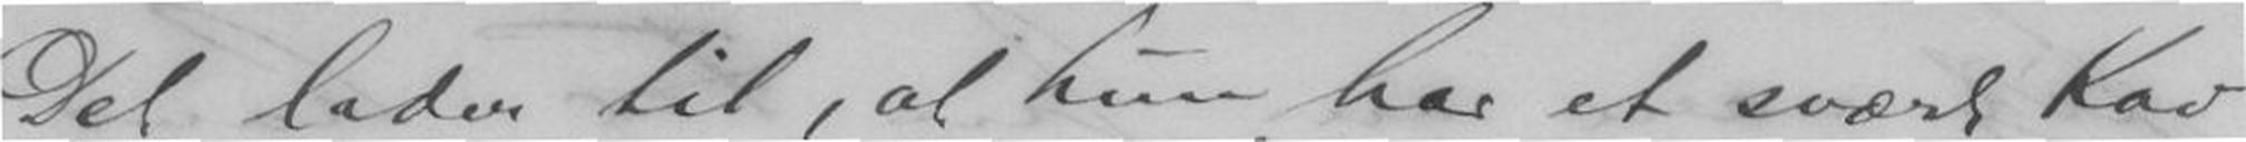Det lader til, at hun har et svært Kav
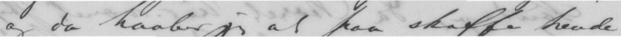og da haaber jeg at faa skaffe hende
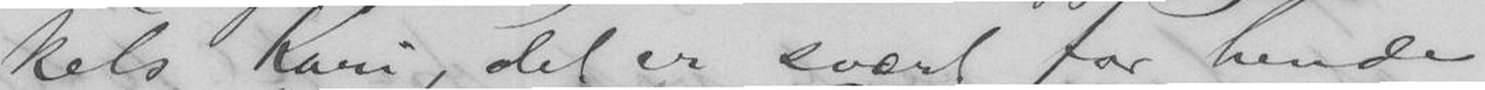kels Kari, det er svært for hende
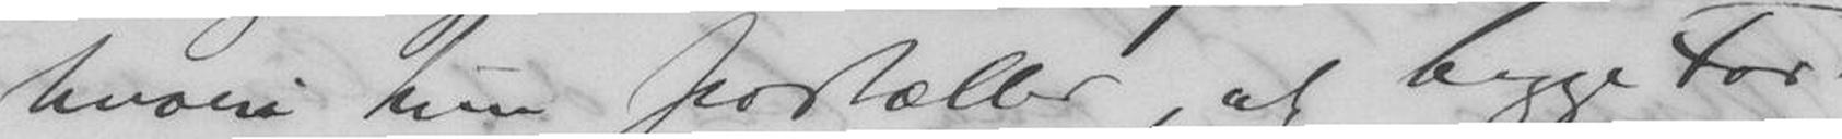hvori hun fortæller, at begge For-
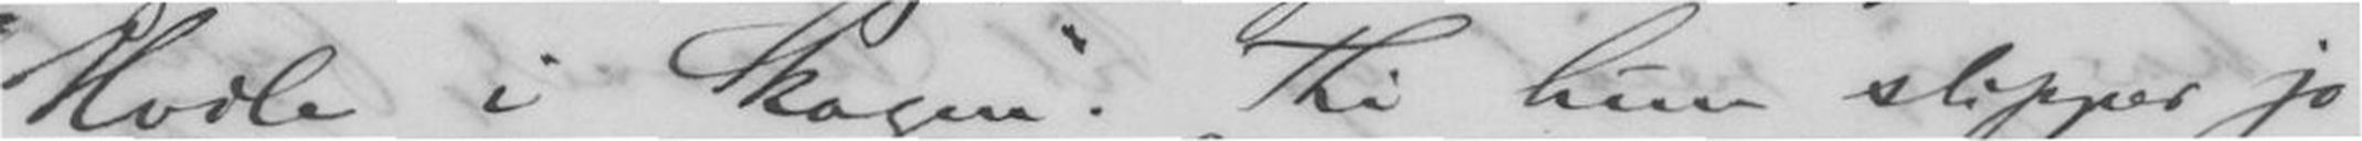Hvile i Skogen. Thi hun slipper jo
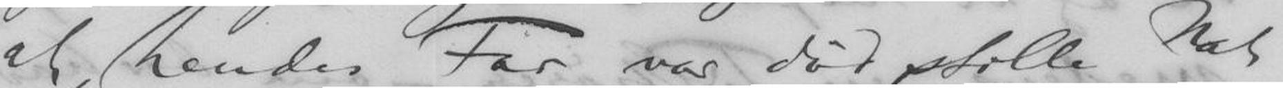at hendes Far var død stille Nat
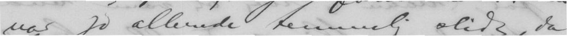var jo allerede temmelig slidt, da
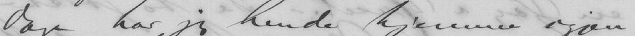Dage har jeg hende hjemme igjen,
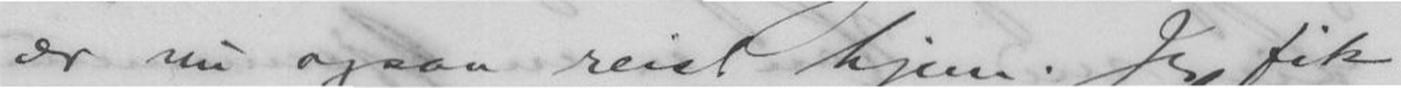er nu ogsaa reist hjem. Jeg fik
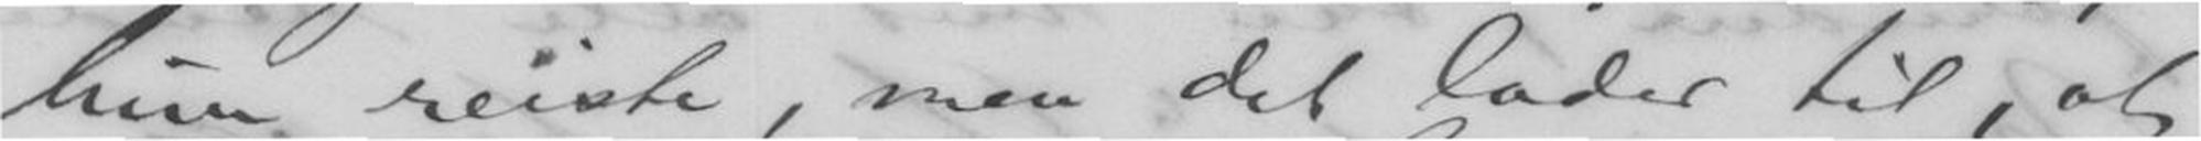hun reiste, men det lader til, at
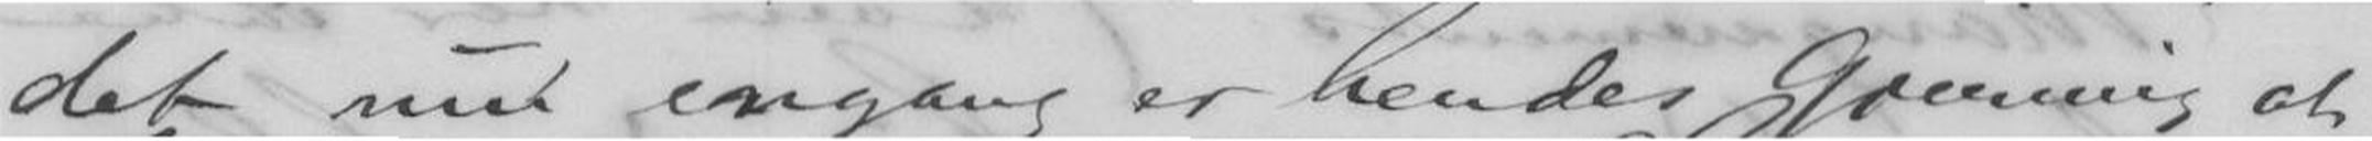det nu engang er hendes gjerning at
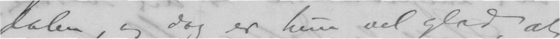dalen, og dog er hun vel glad, at
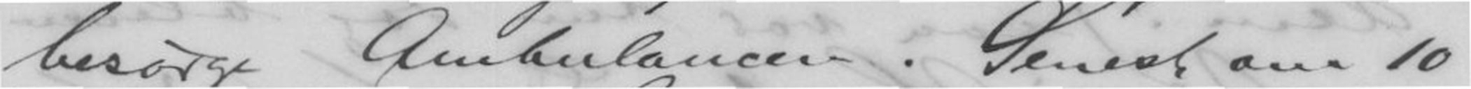besørge Ambulancen. Senest om 10
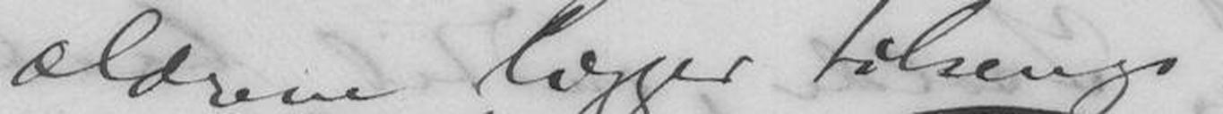ældrene ligger tilsengs
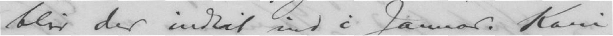blir der indtil ind i Januar. Kari
